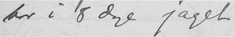har i 8 dage jaget
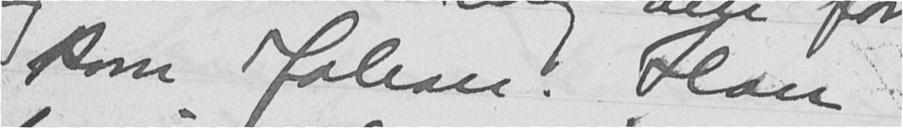kom Johan. Han
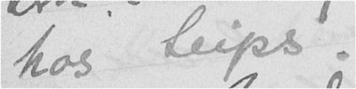hos Seips.
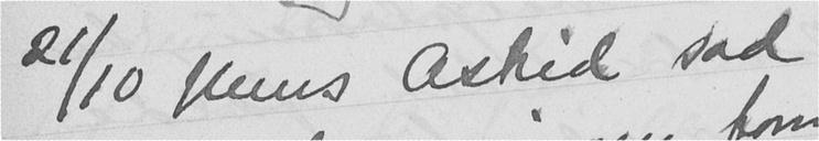21/10 Mens Astrid sad
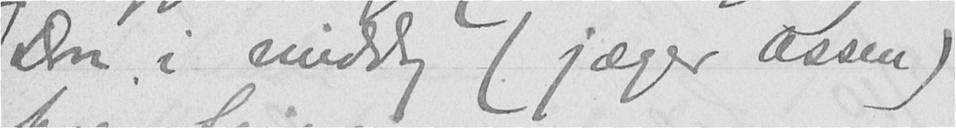Don i middag (jæger Assen)
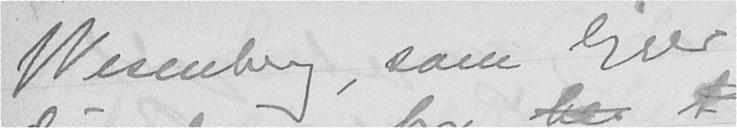Wesenberg, som ligger
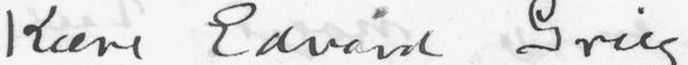Kære Edvard Grieg!
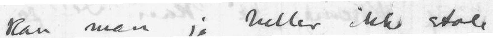kan man jo helle ikke stole
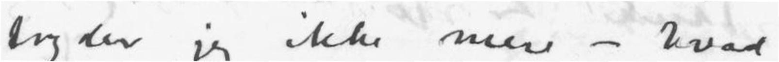bryder jeg ikke mere - hvad
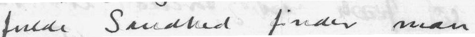fulde Sandhed finder man
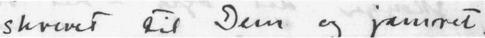skrevet til Dem og jamret.
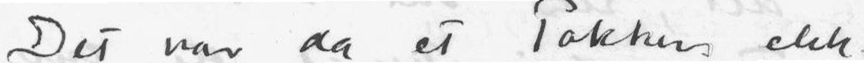Det var da et Takkens elsk-
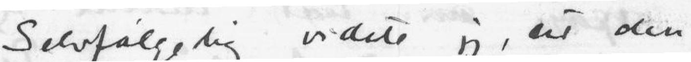Selvfølgelig vidste jeg, det den
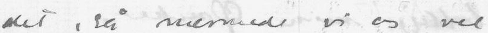det, så nærmed vi os vel
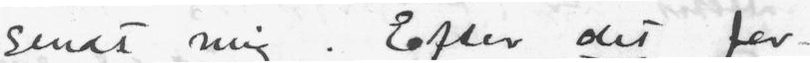sendt mig. Efter det for-
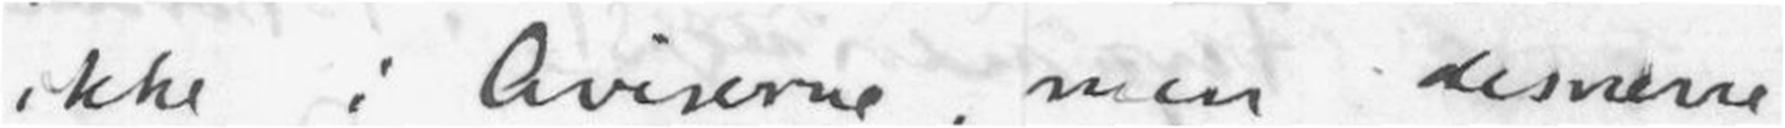ikke i Aviserne, men desværre
